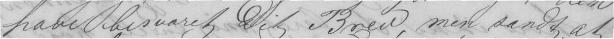have besvaret Dit Brev, men sandt at
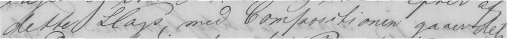dette Slags, med Compositioner gaar det
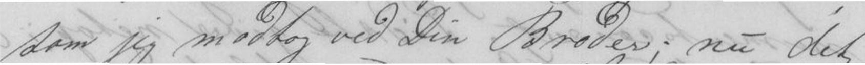som jeg modtog ved Din Broder; nu det
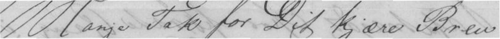Mange Tak for Dit kjære Brev
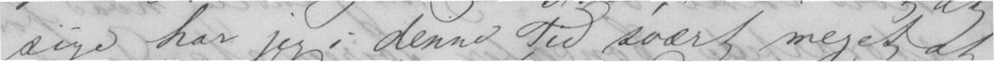sige har jeg i denne Tid svært meget at
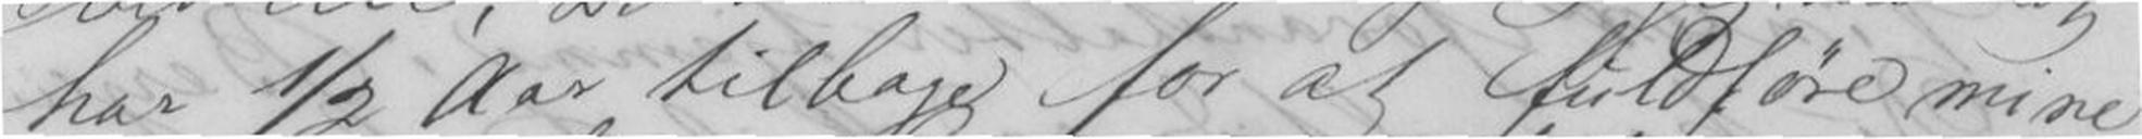har 1/2 Aar tilbage for at fuldføre mine
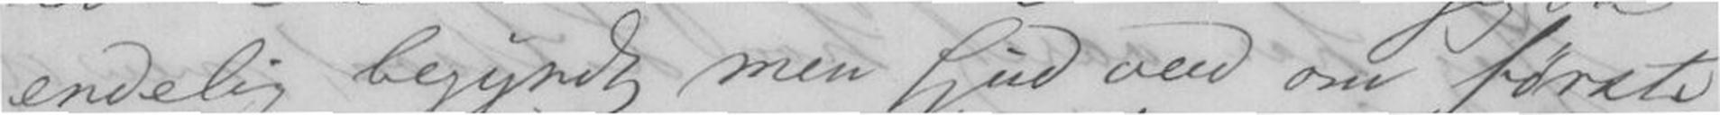endelig begyndt men Gud ved om første
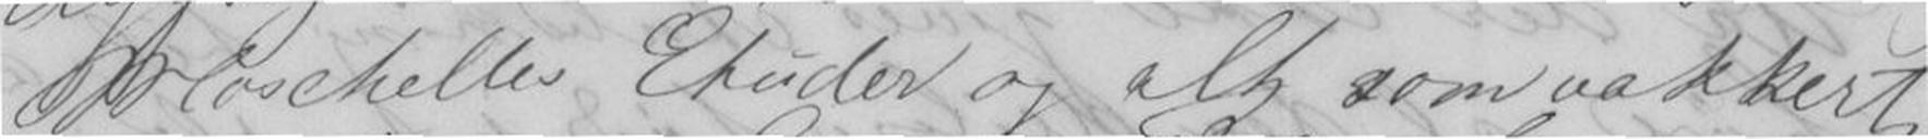Mochella Etuder og alt som vakkert
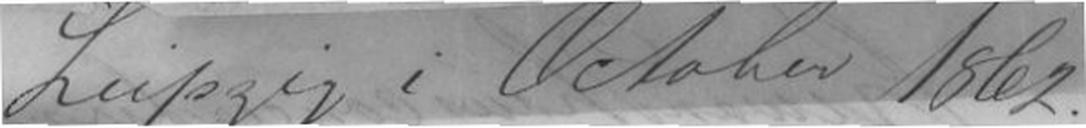Leipzig i October 1862.
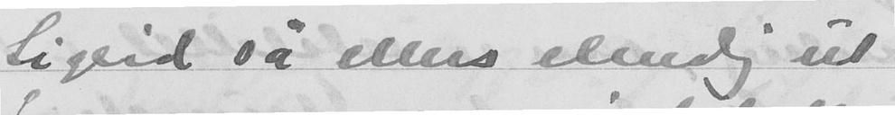Sigrid så ellers elendig ut
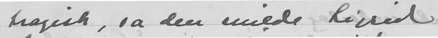tragisk, sa den milde Sigrid
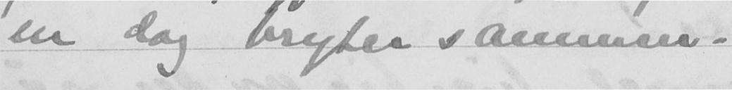en dag bryter sammen.
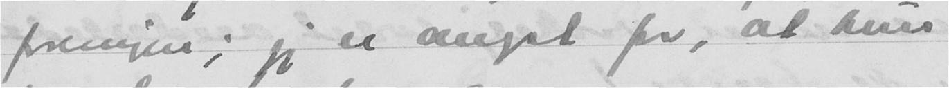foreningen; jeg er angst for, at hun
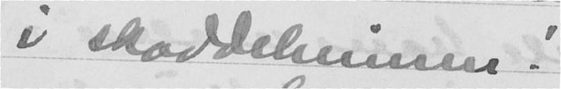i skoddelsnissen!
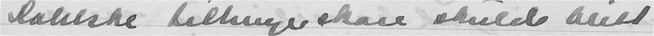Dahlske tilhengerskare skulde blitt
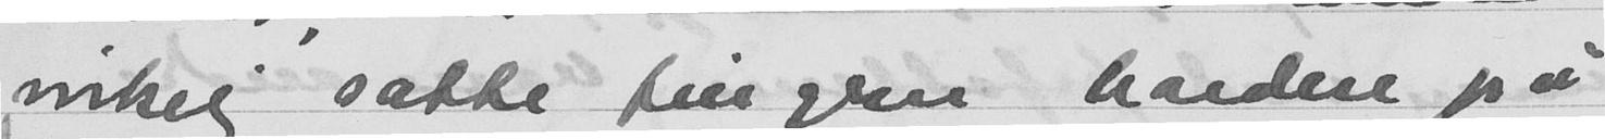virkelig satte fingern hardere på
